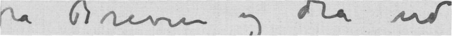på Brevene og dra ud
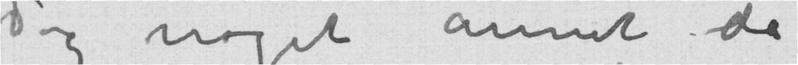dog noget annet da
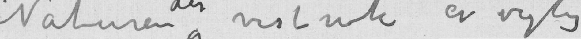Naturen der vistnok er vigtig
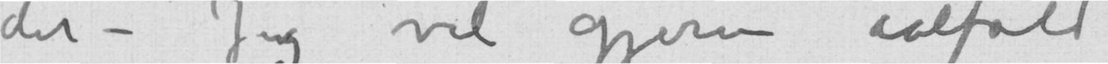det – Jeg vil gjerne ialfald
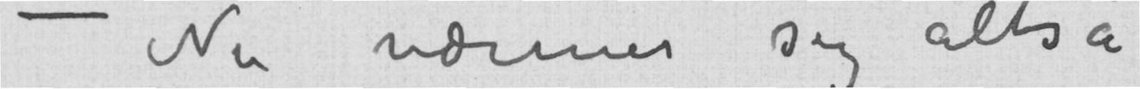– Nu nærmer sig altså
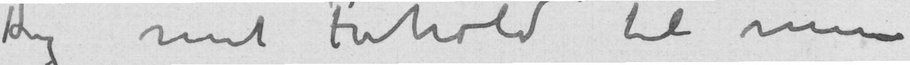Dig mit Forhold til mine
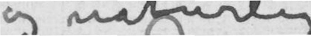og naturlig
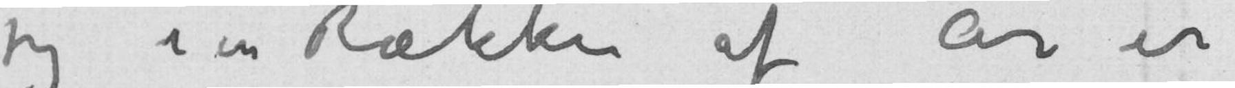jeg i en Række af År er
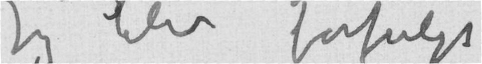Jeg blev forfulgt
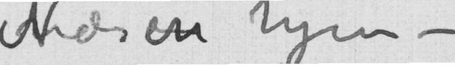Næsen hjem –
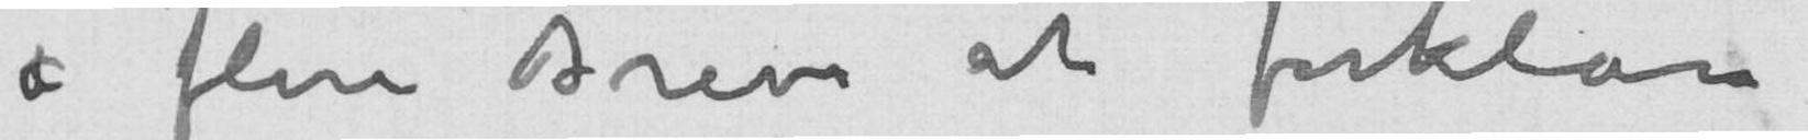i flere Breve at forklare
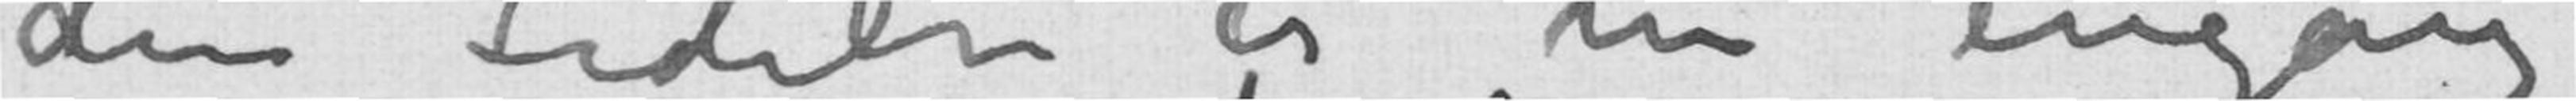den Lidelse er nu engang
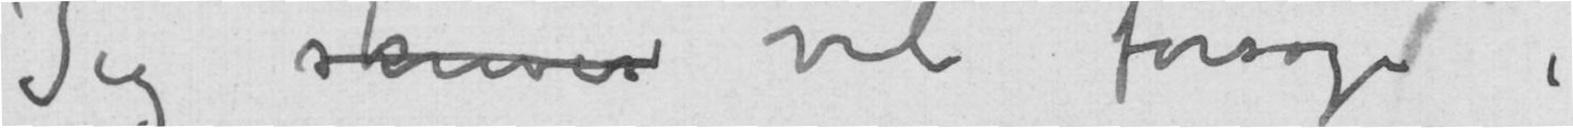Jeg skriver vil forsøge
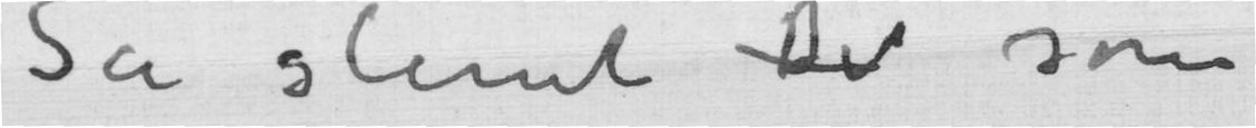Så slemt D som
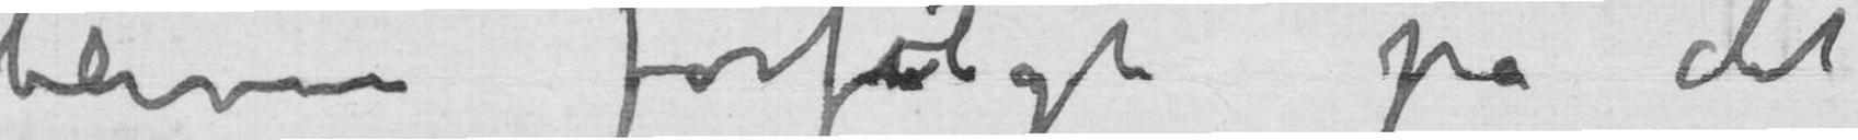bleven forfulgt på det
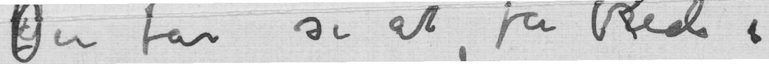Du får se at få Rede i
